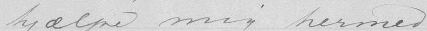hjælpe mig hermed,
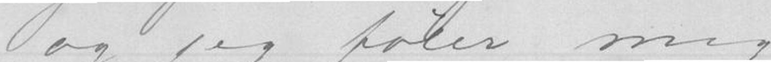og jeg føler mig
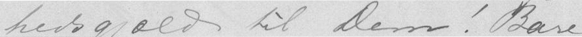hedsgjæld til Dem! Bare
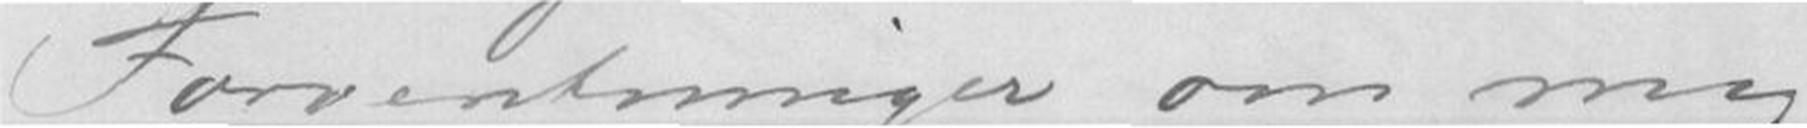Forventninger om mig!
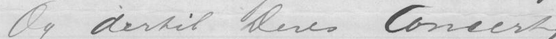Og dertil Deres Concert,
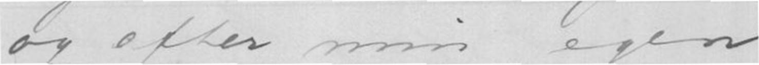og efter min egen
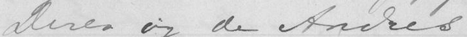Deres og de Andres
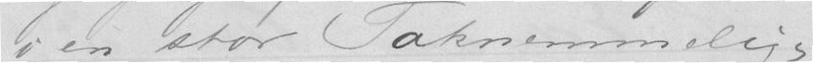i en stor Taknemmelig-
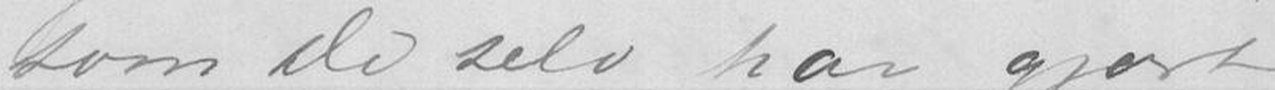som De selv har gjort
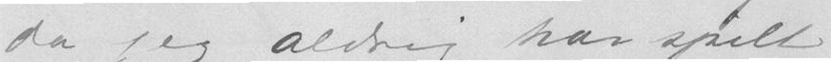da jeg aldrig har spilt
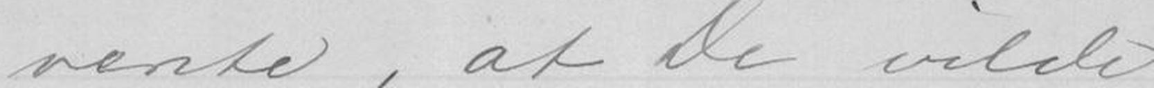vente, at De vilde
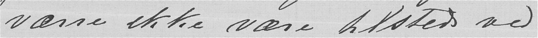værre ikke være tilstede ved
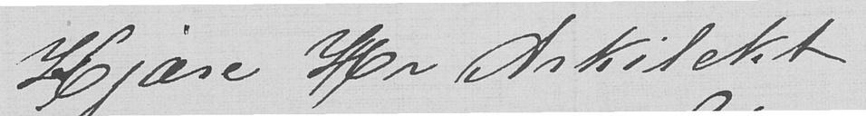Kjære Hr Arkitekt
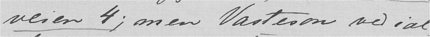veien 4; men Vasteson ved ial-
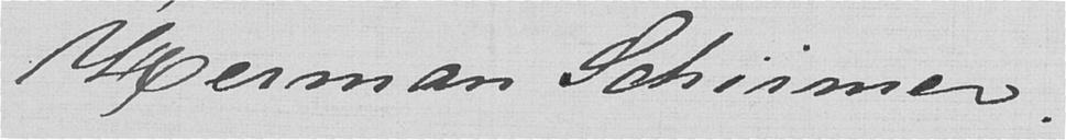Herman Schirmer.
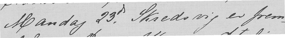Mandag 23de. Skredsvig er frem-
